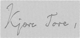Kjære Tore,
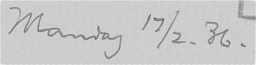Mandag 17/2. 36.
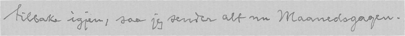tilbake igjen, saa jeg sender alt nu Maanedsgagen.
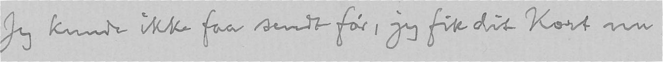Jeg kunde ikke faa sendt før, jeg fik dit Kort nu
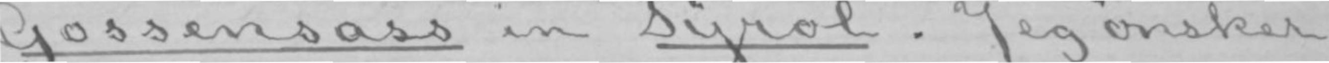Gossensass in Tyrol. Jeg ønsker
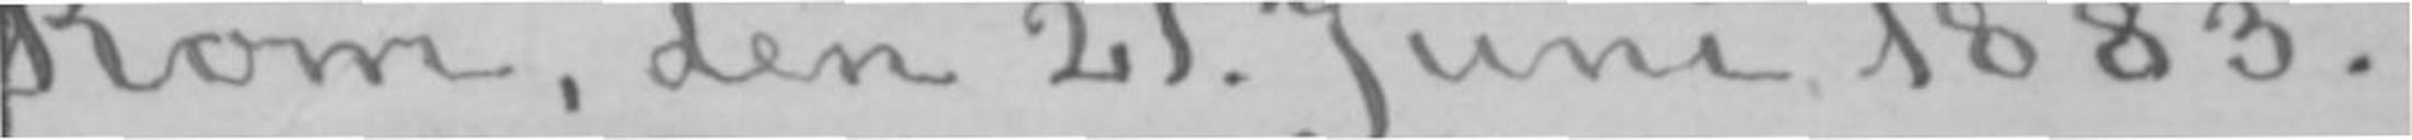Rom, den 21. Juni 1883.
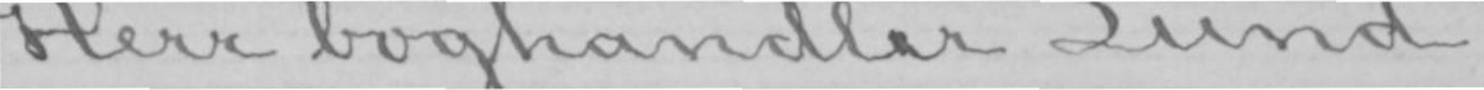Herr boghandler Lund!
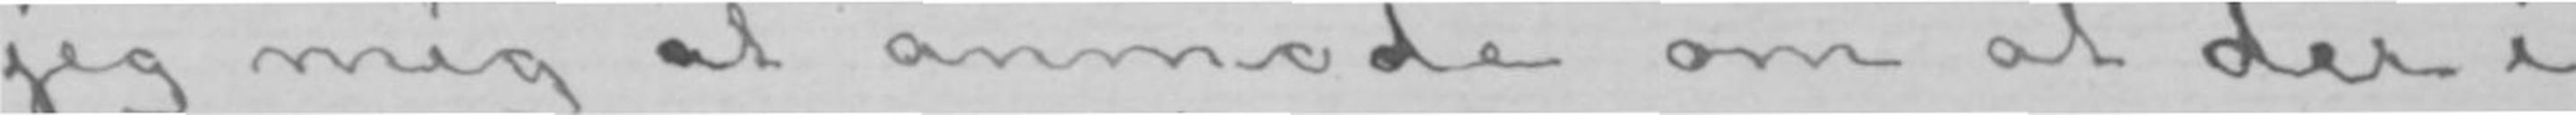jeg mig at anmode om at der i
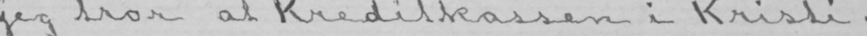jeg tror at Kreditkassen i Kristi-
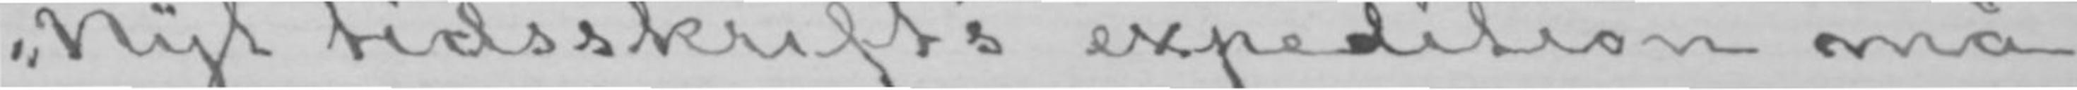«Nyt tidsskrift»s expedition må
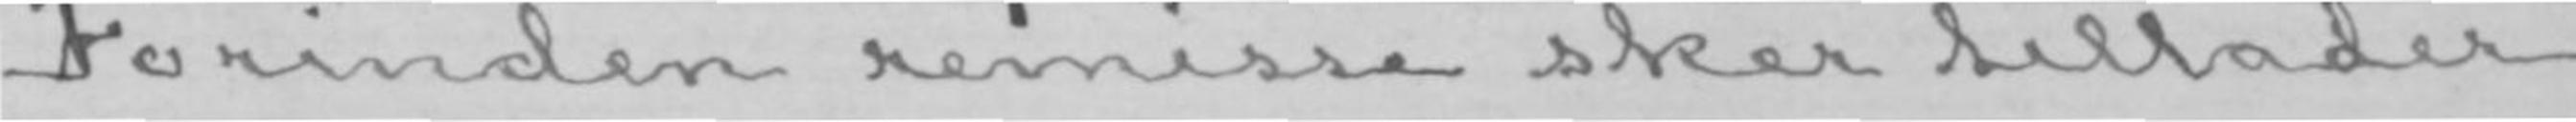Forinden remisse sker tillader
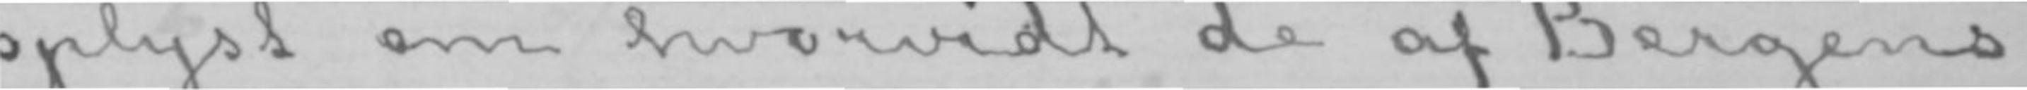oplyst om hvorvidt de af Bergens
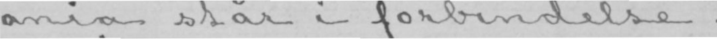ania står i forbindelse.
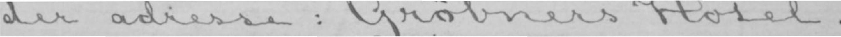der adresse: Gröbners Hotel.
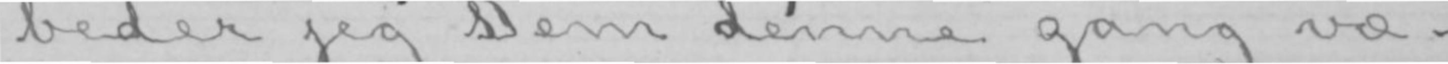beder jeg Dem denne gang væ-
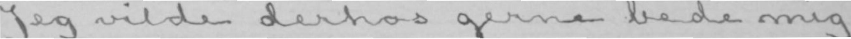Jeg vilde derhos gerne bede mig
